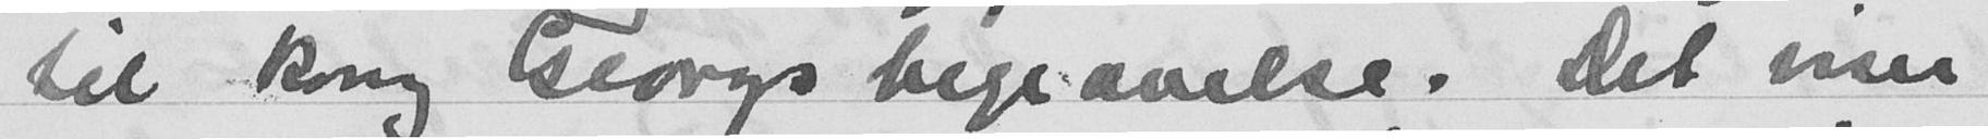til kong Georgs begravelse. Det viser
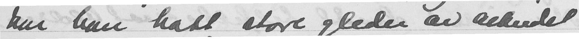har han hatt store gleder av arbeidet
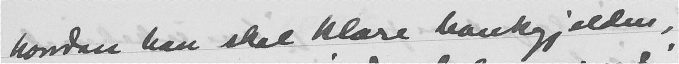hvordan han skal klare bankgjelden,
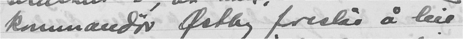kommandør Østberg foreslår å leie
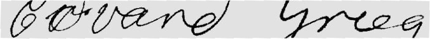Edvard Grieg!
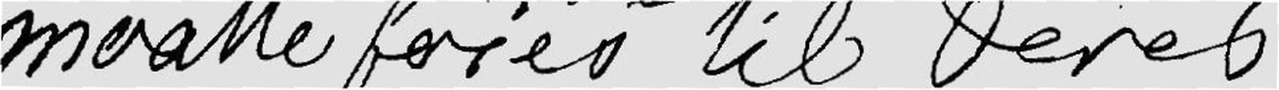maatte føies til Deres
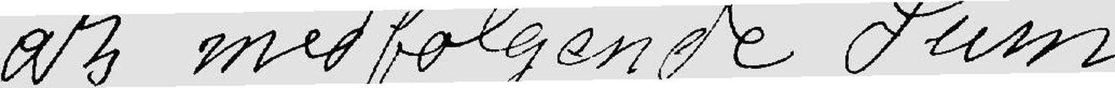at medfølgende Sum
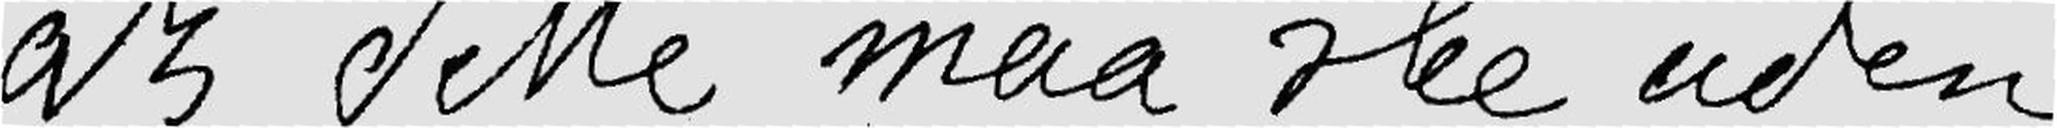at dette maa ske uden
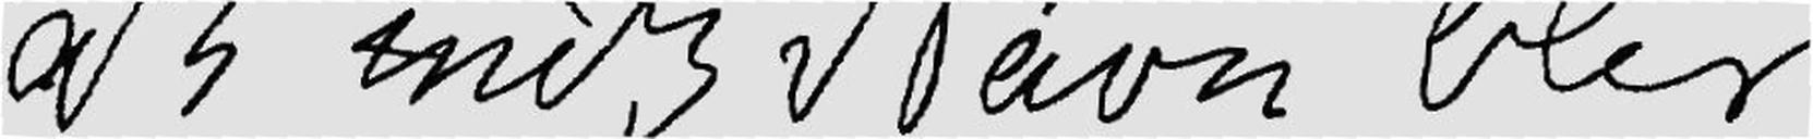at mit Navn blir
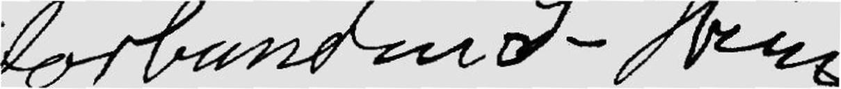Forbunden S- Ibsen
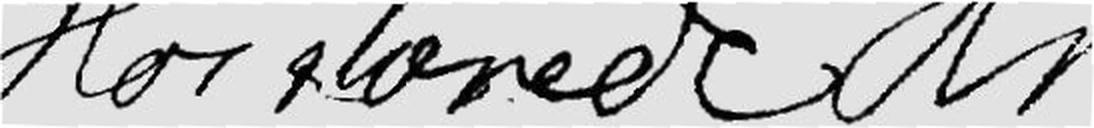Høistærede Hr
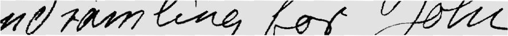Indsamling for John
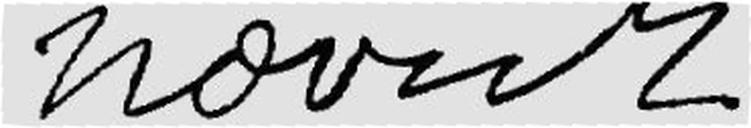nævnt.
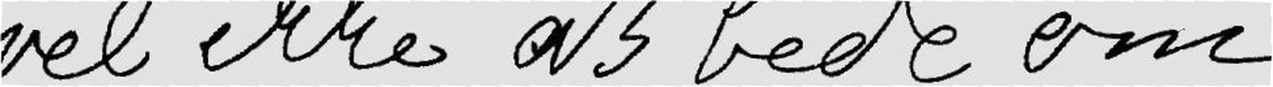vel ikke at bede om,
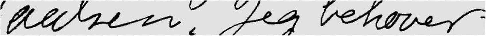Paulsen. Jeg behøver
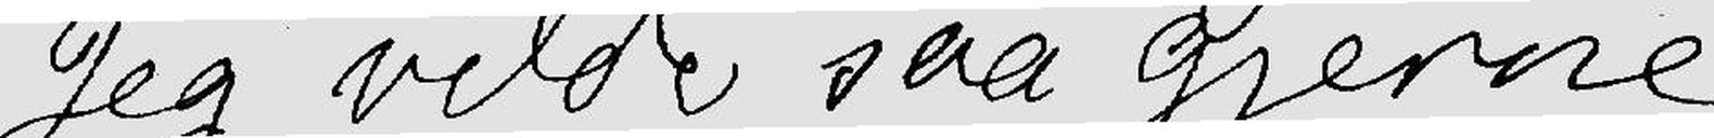Jeg vilde saa gjerne
